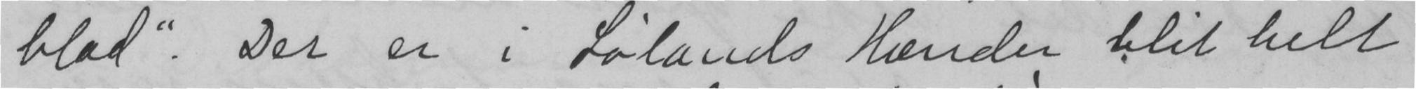blad". Der er i Lølands Hænder blit helt
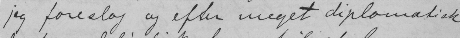jeg foreslog og efter meget diplomatisk
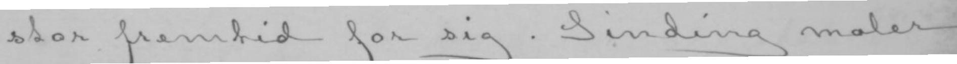stor fremtid for sig. Sinding maler
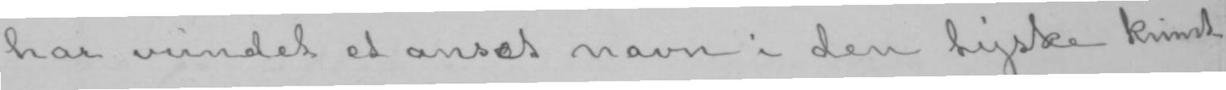har vundet et anset navn i den tyske kunst-
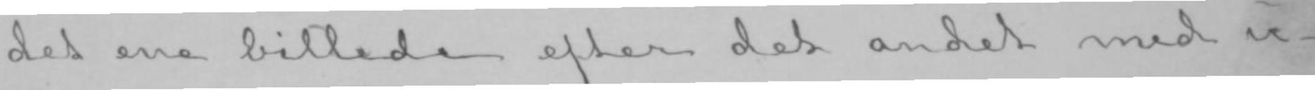det ene billede efter det andet med u-
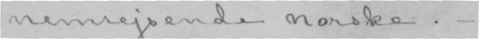nemrejsende norske.-
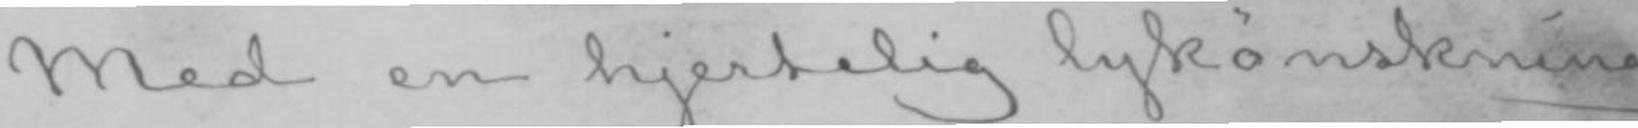Med en hjertelig lykønskning
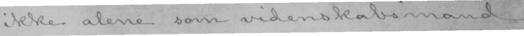ikke alene som videnskabsmand
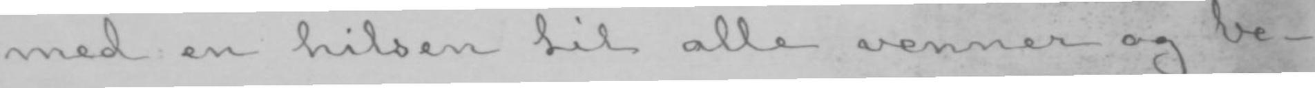med en hilsen til alle venner og be-
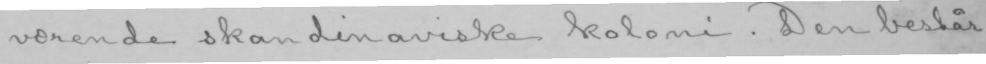værende skandinaviske koloni. Den består
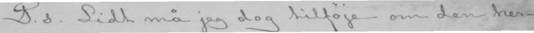P. s. Lidt må jeg dog tilføje om den her-
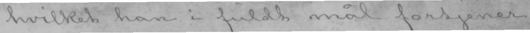hvilket han i fuldt mål fortjener,
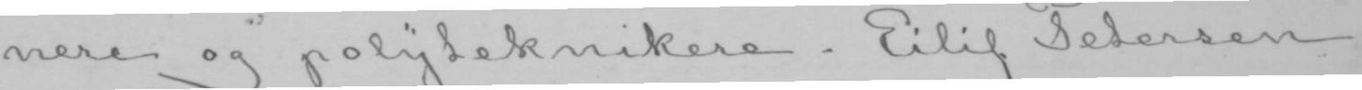nere og polyteknikere. Eilif Petersen
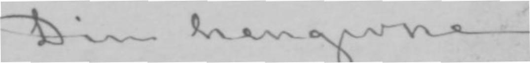Din hengivne
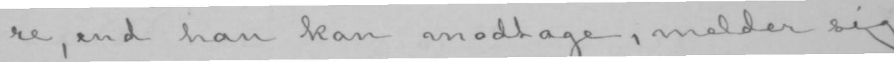re, end han kan modtage, melder sig
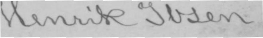Henrik Ibsen.
